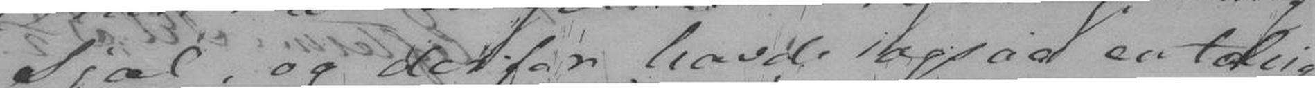Sjæl, og derfor havde ogsaa en talrig
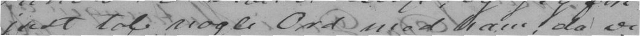just tale nogle Ord med ham, da vi
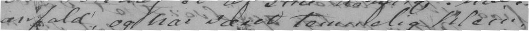andfald, og har været temmelig klein,
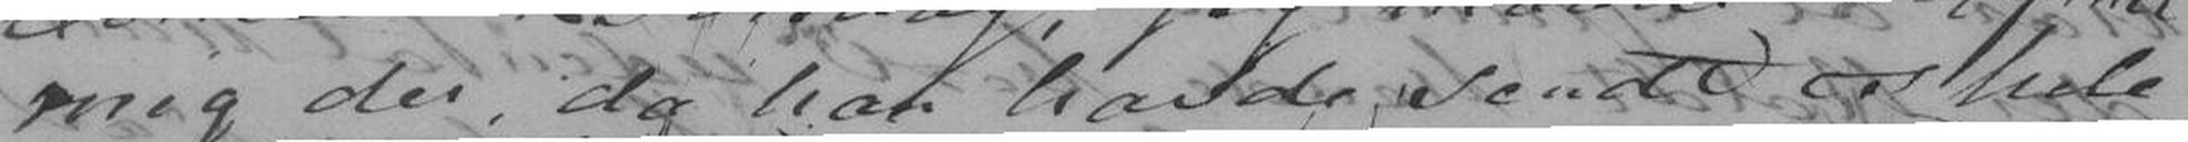mig der, da han havde sendt os hele
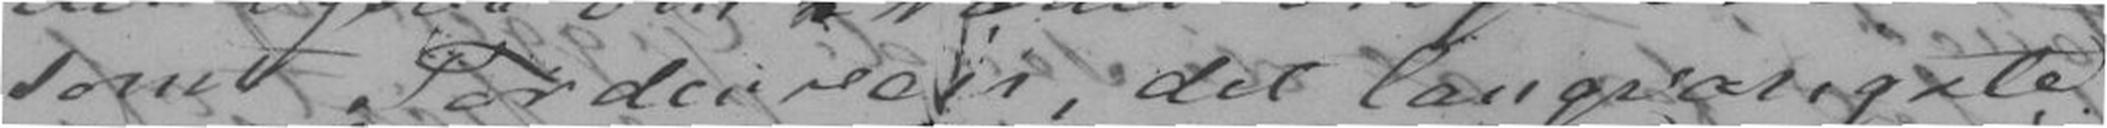somt Tordenveir; det langvarigste
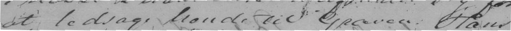at ledsage hende til Graven. Hans
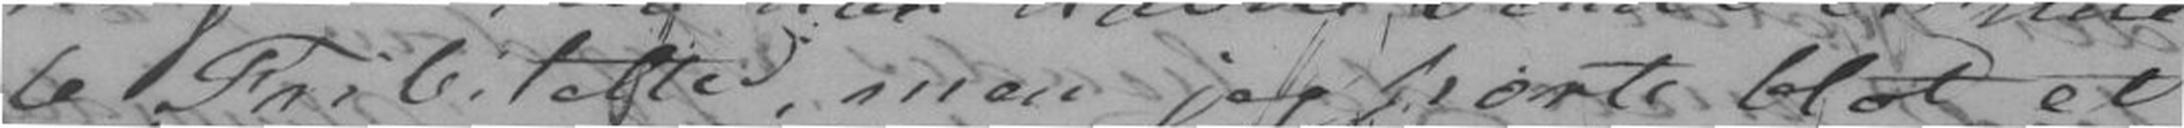6 Fribiletter, men jeg hørte blot et
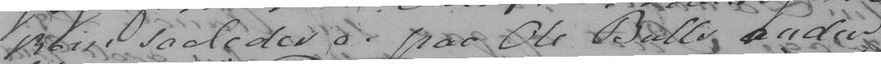kom saaledes ei paa Ole Bulls anden
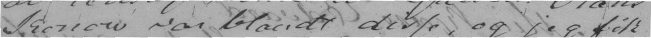Konow var blandt disse, og jeg fik
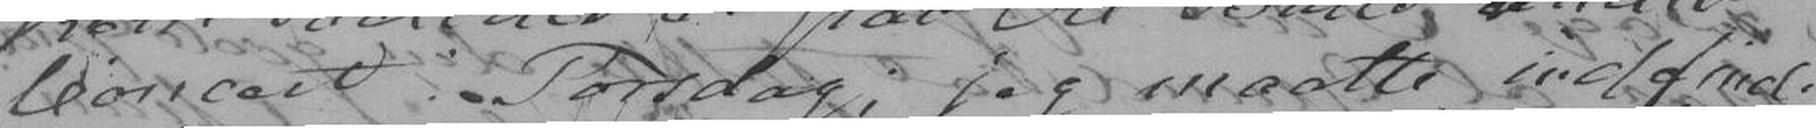Concert Torsdag, jeg maatte indfinde
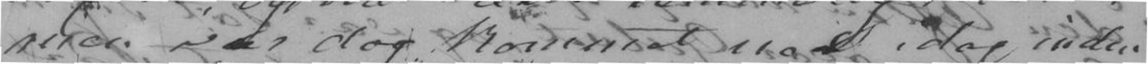men var dog kommet ned idag inden
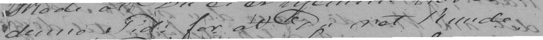denne Tid, for at Du ret kunde
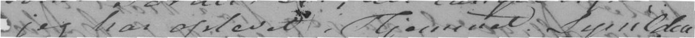jeg har oplevet. Hjemmet Lynilden
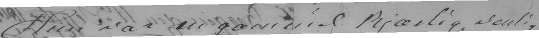Hun var en gammel kjærlig venlig
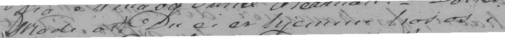Skade at Du ei er hjemme hos os i
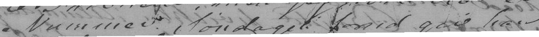Nummer. Søndagen forrud gav han
